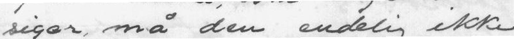siger, må den endelig ikke
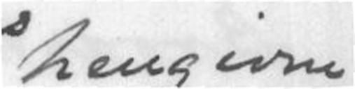hengivne
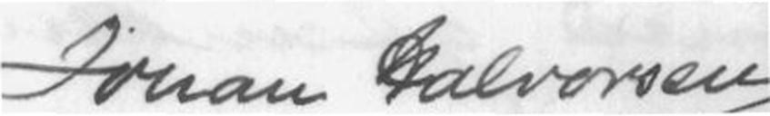Johan Halvorsen
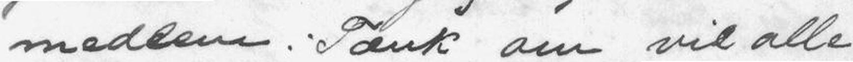medlem. Tænk om vil alle
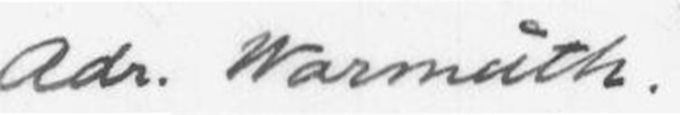Adr. Warmuth
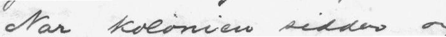Når kolonien sidder og
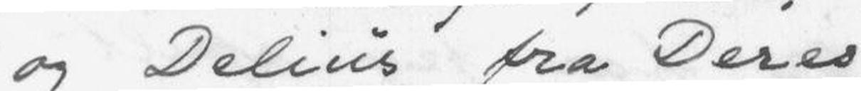og Delius fra Deres
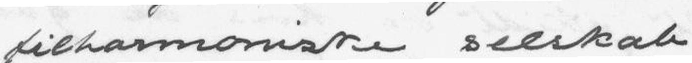filharmoniske selskab
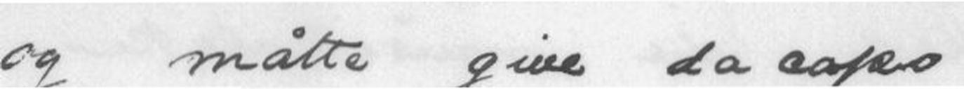og måtte give da capo.
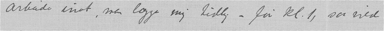arbeide inat, men lægge mig tidlig – før kl. 1, saa vilde
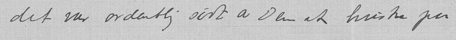det var ordentlig sødt av Dem at huske paa
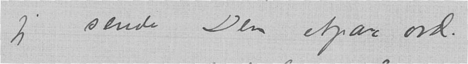jeg sende Dem etpar ord.
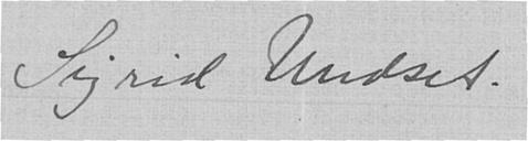Sigrid Undset.
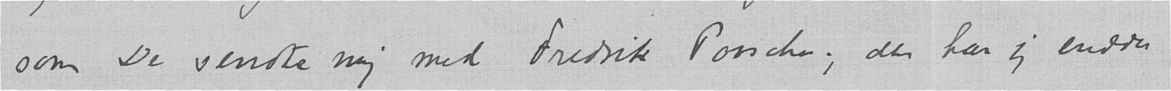som De sendte mig med Fredrik Paasche; den har jeg endda
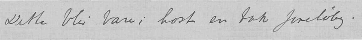Dette blir bare i hast en tak foreløbig.
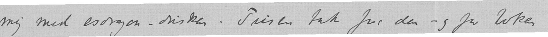mig med esdragon-dusken. Tusen tak for den – og for boken
